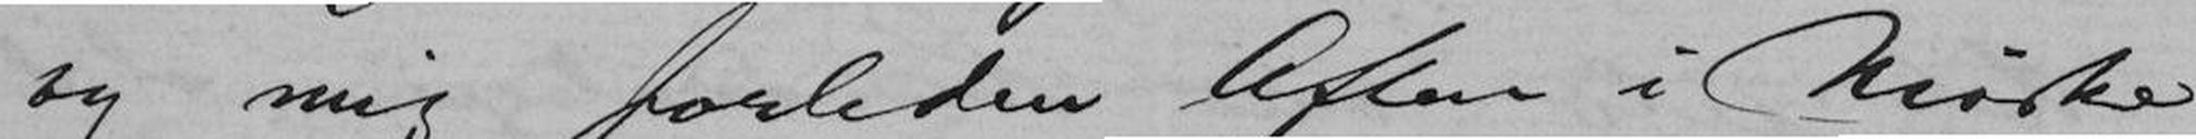og mig forleden Aften i Mørke
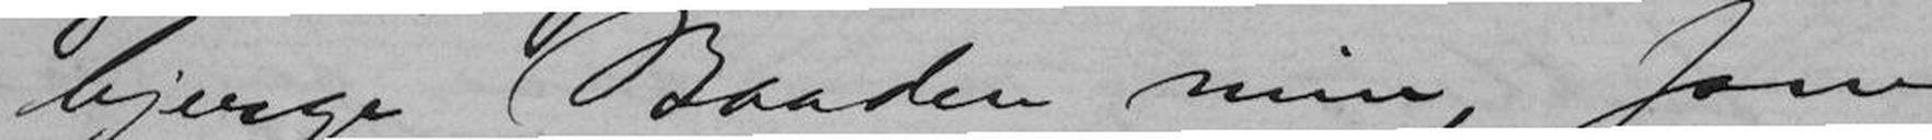bjerge Baaden min, som
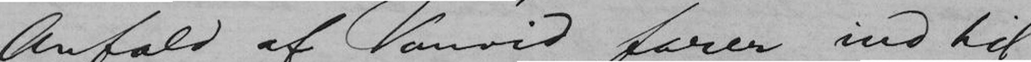Anfald af Vanvid farer ind til
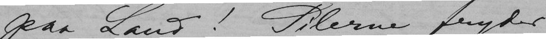paa Land! Pilerne fryder
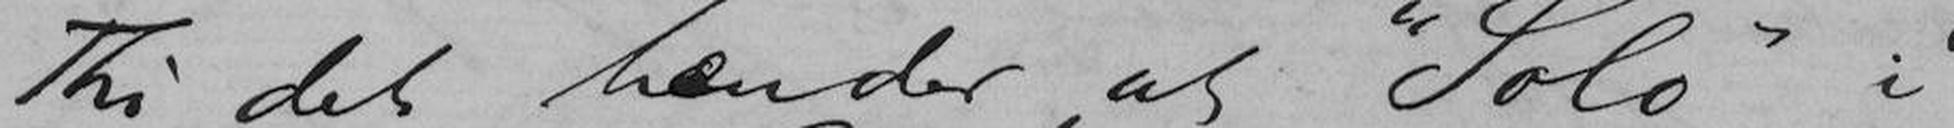Thi det hænder, at "Solo" i
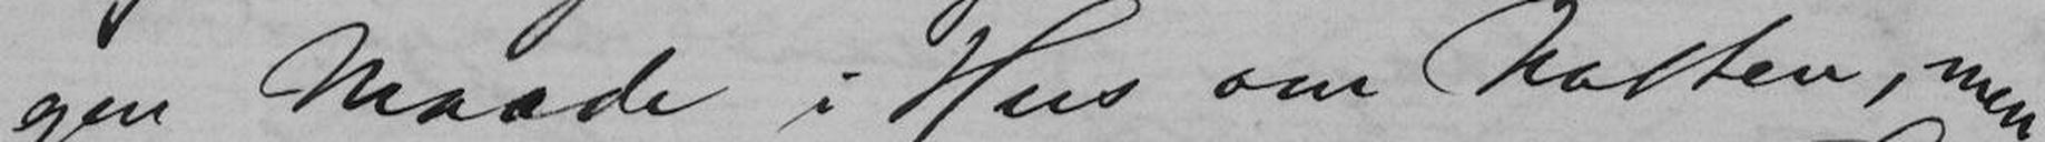gen Maade i Hus om natten, men
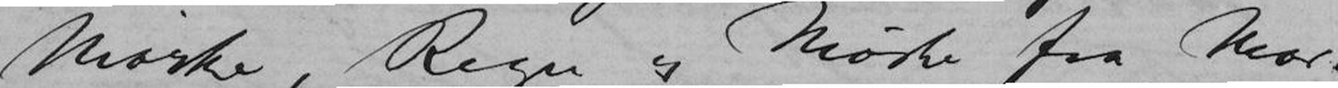Mørke, Regn og Mørke fra Mor-
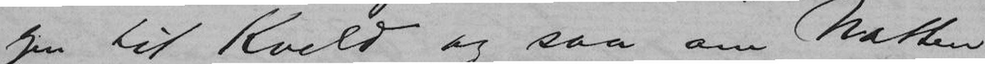gen til Kveld og saa om Natten
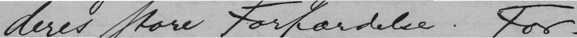deres store Forfærdelse. For-
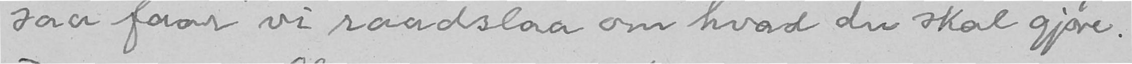saa faar vi raadslaa om hvad du skal gjøre.
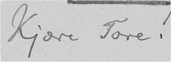Kjære Tore!
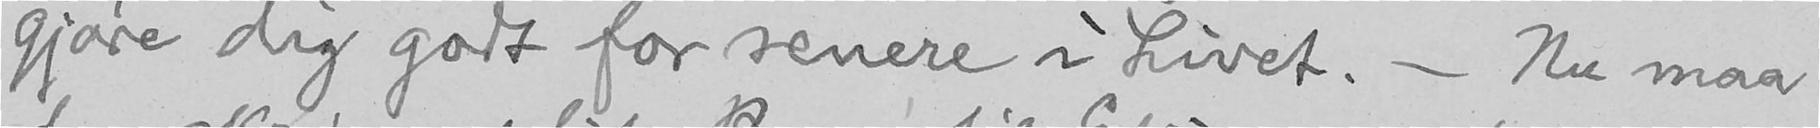gjøre dig godt for senere i Livet. - Nu maa
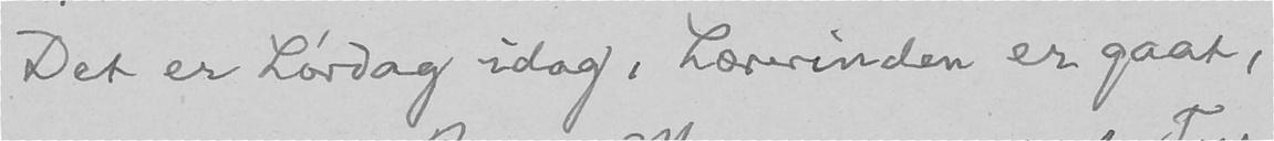Det er Lørdag idag, Lærerinden er gaat,
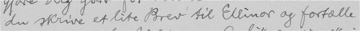du skrive et lite Brev til Ellinor og fortælle
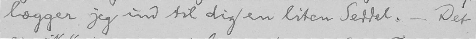lægger jeg ind til dig en liten Seddel. - Det
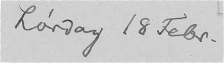Lørdag 18 Febr.
